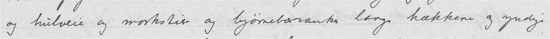og hulveie og markstier og bjørnebærranker langs hækkene og yndige
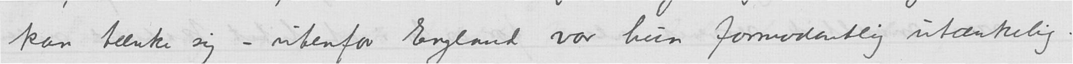kan tænke sig - utenfor England var hun formodentlig utænkelig.
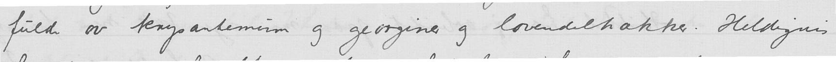fulde av krysantemum og georginer og lavendelhækker. Heldigvis
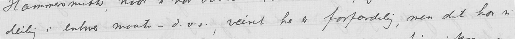deilig i enhver maate - d.v.s., veiret her er forfærdelig, men det har vi
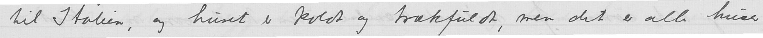til Italien, og huset er koldt og trækfuldt, men det er alle huse
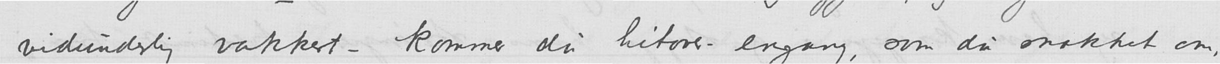vidunderlig vakkert - kommer du hitover engang, som du snakket om,
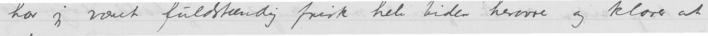har jeg været fuldstændig frisk hele tiden herovre og klarer at
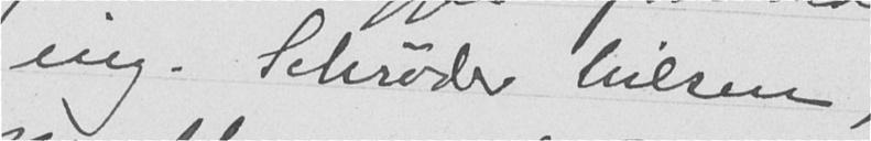ing. Schrøder Nilsen,
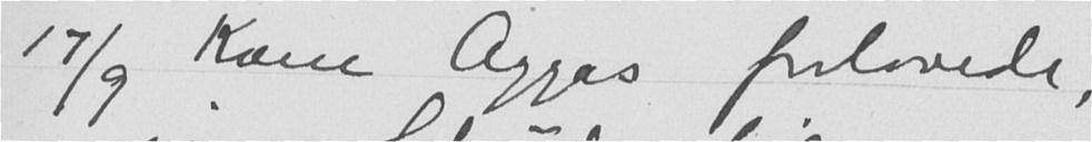17/9 Kom Aggas forlovede,
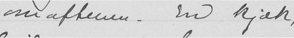om aftenen. En kjæk,
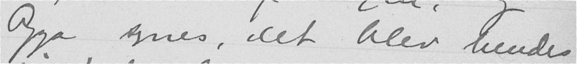Agga synes, det blev hendes
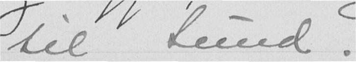til Sund.
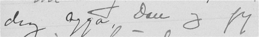drog Agga, Don og jeg
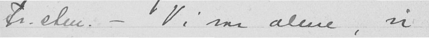Fr. sten. - Vi var alene, vi
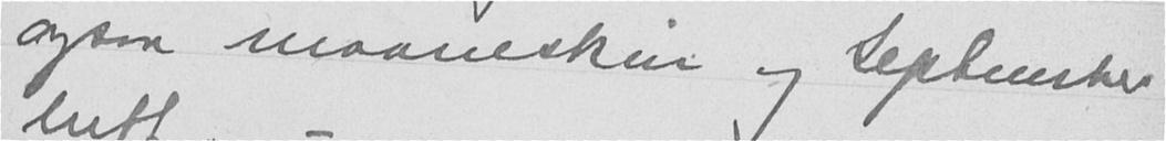ogsaa maaneskin og September-
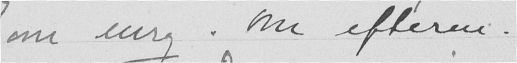om mrg. Om efterm.
